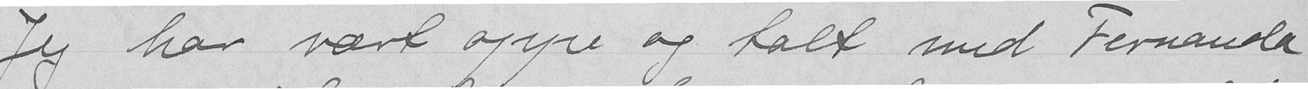Jeg har være oppe og talt med Fernanda
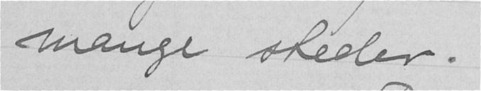mange steder.
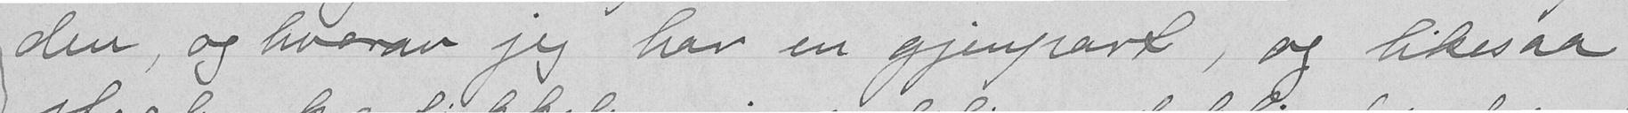den, og hvorav jeg har en gjenpart, og likesaa
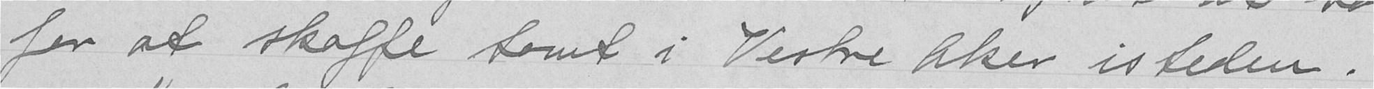for at skaffe tomt i Vestre Aker isteden.
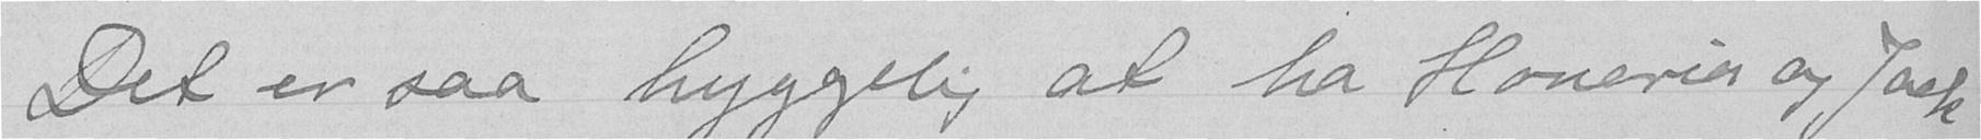Det er saa hyggelig at ha Honeria og Jack
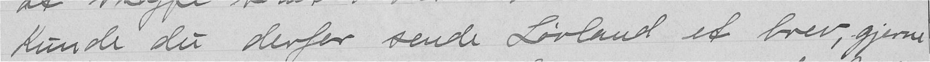Kunde du derfor sende Løvland et brev, gjerne
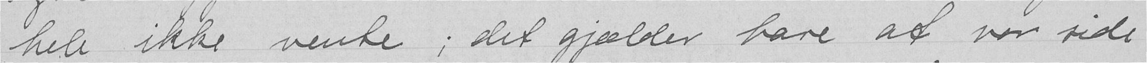hele ikke vente; det gjælder bare at vor side
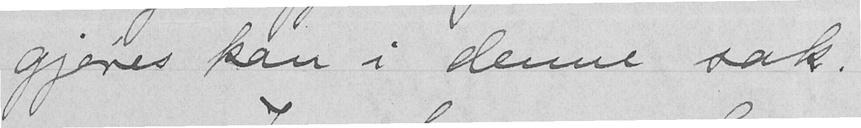gjøres kan i denne sak.
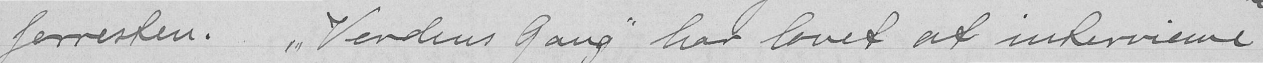forresten. Verdens Gang har lovet at interviewe
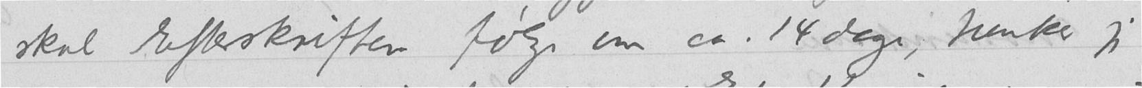skal Efterskriften følge om ca. 14 dage, tænker jeg
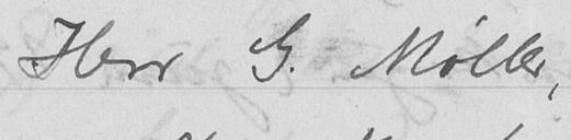Herr G. Møller,
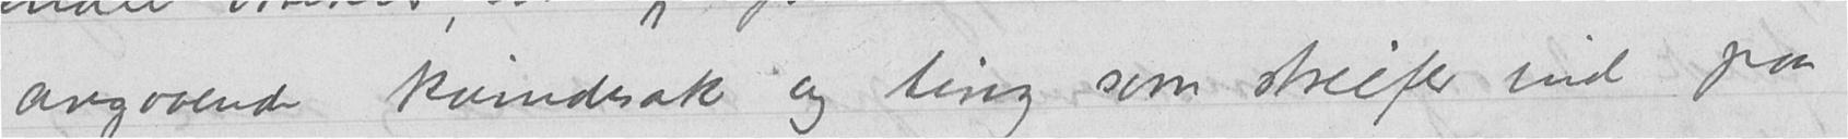angaaende kvindesak og ting som streifer ind paa
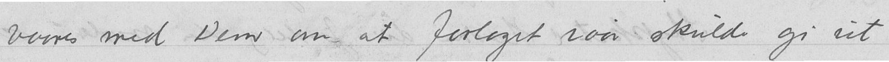vaares med Dem om at forlaget iaar skulde gi ut
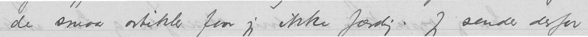de smaa artikler faar jeg ikke færdig. Jeg sender derfor
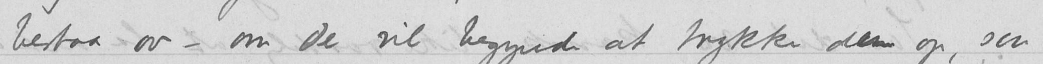bestaa av – om De vil begynde at trykke dem op, saa
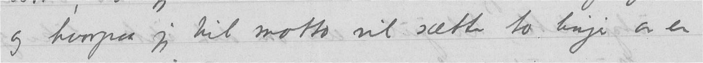og hvorpaa jeg til motto vil sætte to linjer av en
